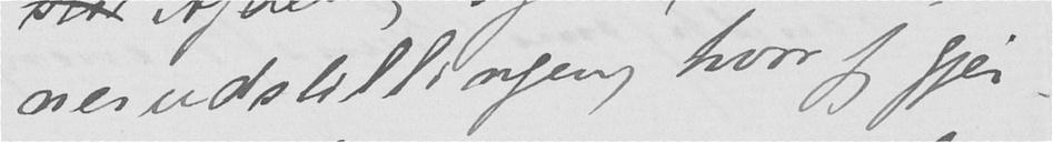nerudstillingen, hvor jeg gjer-
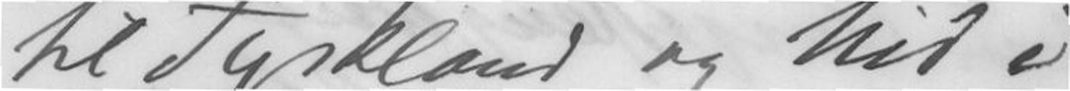til Tyskland og hid i
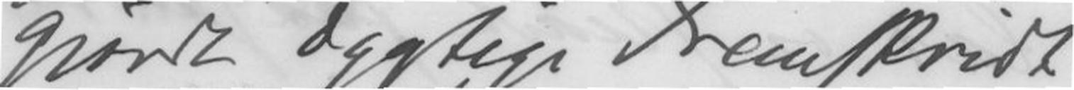gjordt dygtige Fremskridt
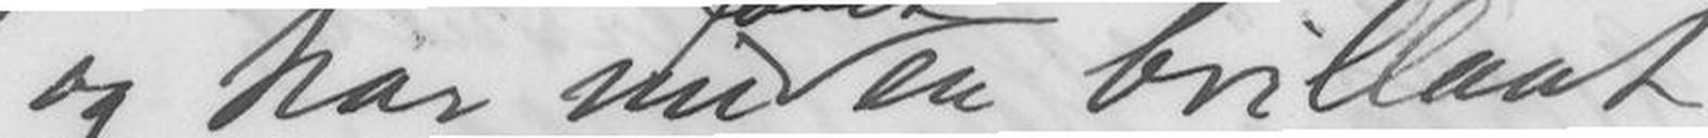og har nu en brillant
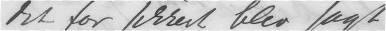det for sikkert blev sagt
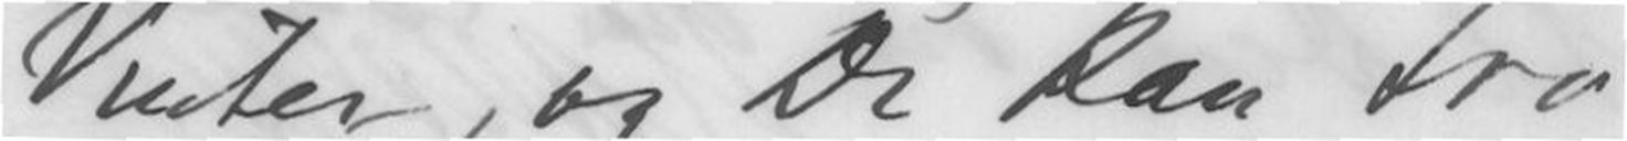Vinter, og De kan tro
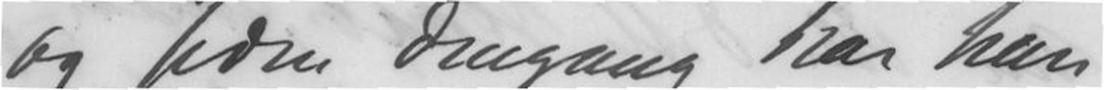og siden dengang har han
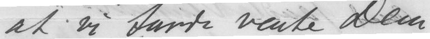at vi turde vente Dem
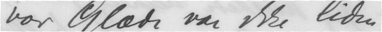vor Glæde var ikke liden.
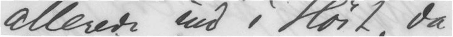allerede ind i Høst, da
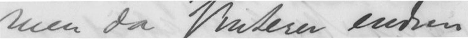men da Vinteren endnu
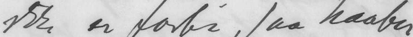ikke er forbi, saa haaber
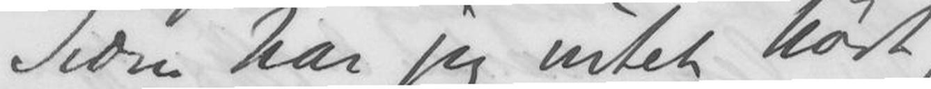Siden har jeg intet hørt,
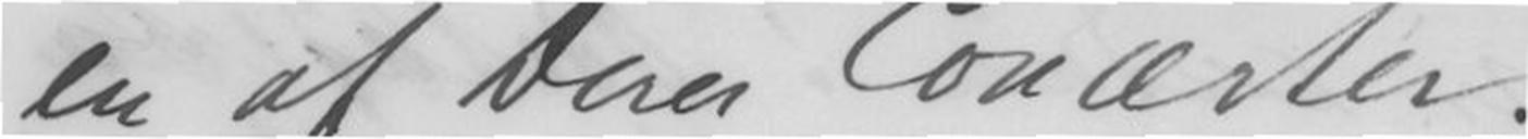en af Deres Concerter;
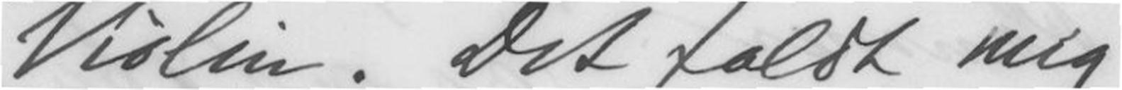Violin. Det faldt mig
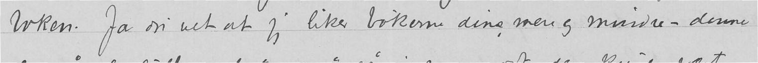boken. Ja du vet at jeg liker bøkerne dine mere og mindre – denne
